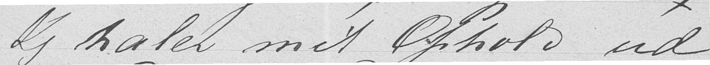Jeg haler mit Ophold ud
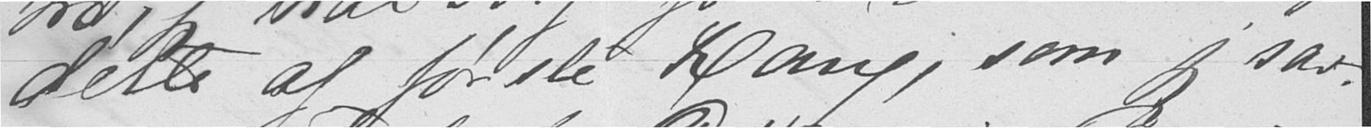dette af første Rang, som jeg sav-
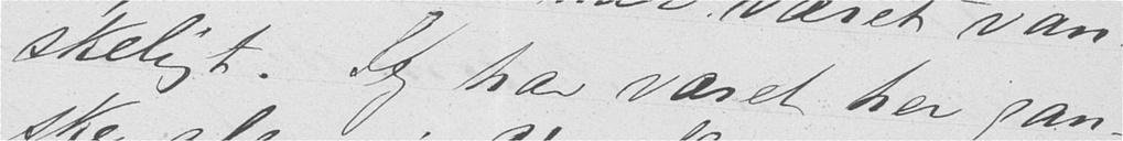skeligt. Jeg har været her gan-
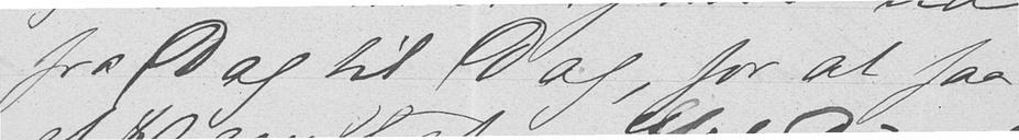fra Dag til Dag, for at faa
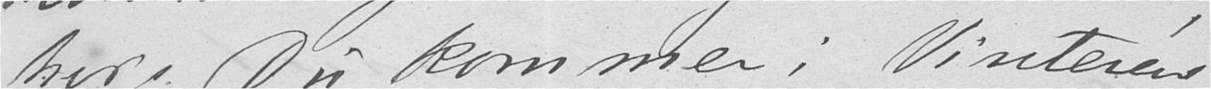hvis Du kommer i Vinterens
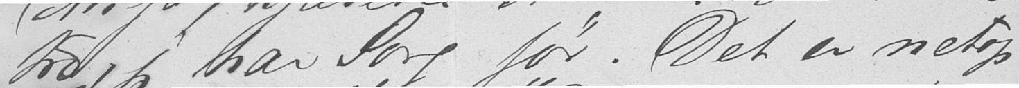tro, jeg har Sorg før. Det er netop
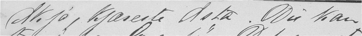Akja, kjæreste Asta. Du kan
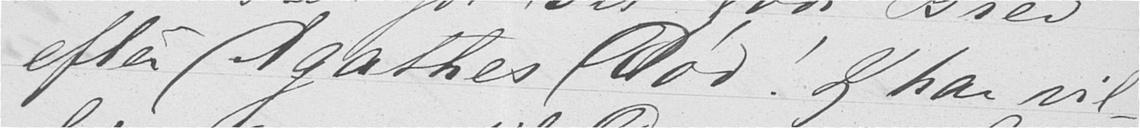efter Agathes Død! Jeg har vil-
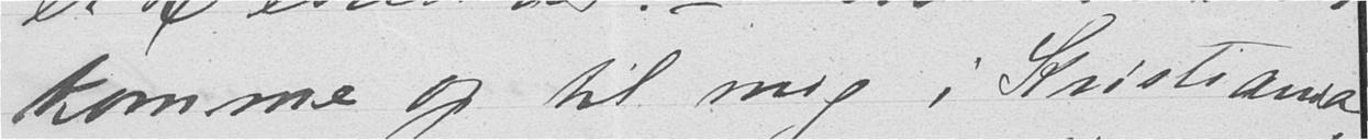komme op til mig i Kristiania,
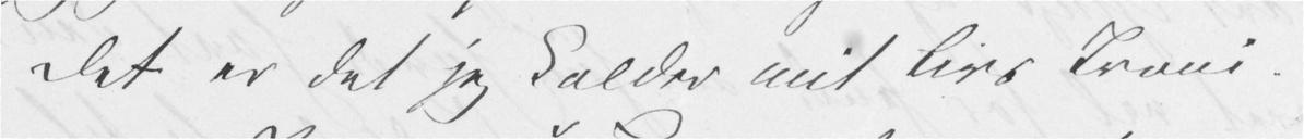Det er det jeg kalder mit Livs Ironi.
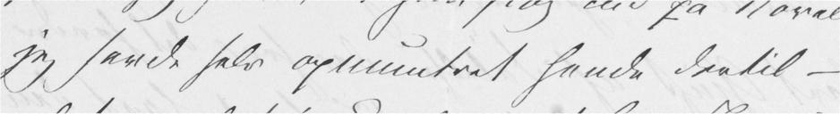jeg havde selv opmuntret hende dertil –
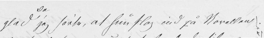glad jeg hørte, at hun slog ind på Novellen
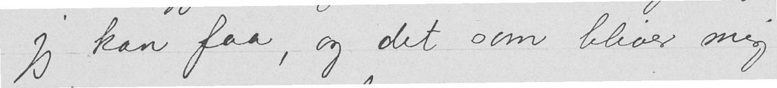jeg kan faa, og det som bliver mig
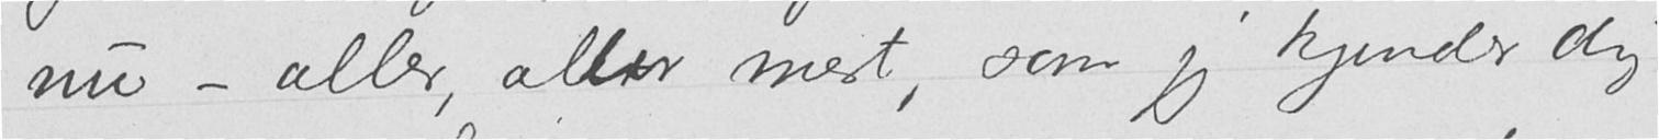nu - aller, aller mest, som jeg kjender dig
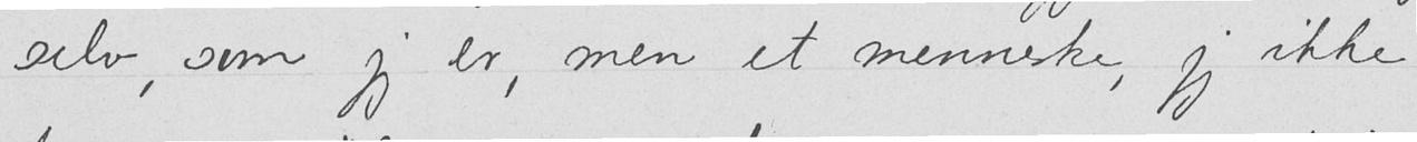selv, som jeg er, men et menneske, jeg ikke
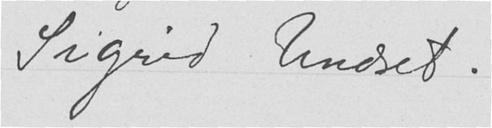Sigrid Undset.
